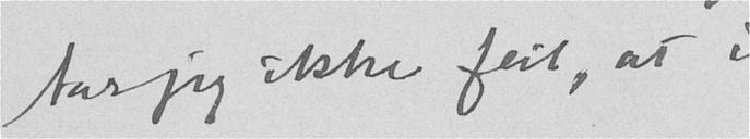tar jeg ikke feil, at
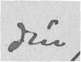din
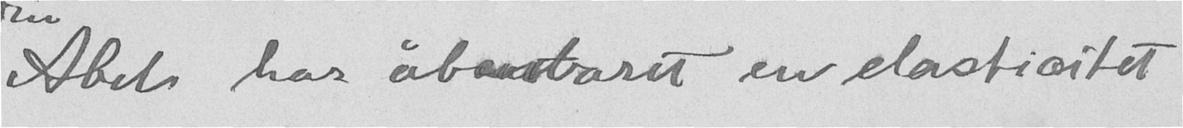Abel har åbenbaret en elasticitet.
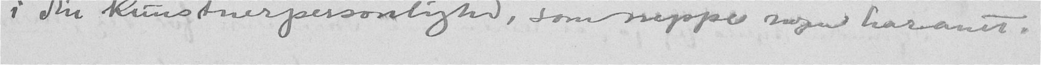i din kunstnerpersonlighed, som neppe nogen har anet.
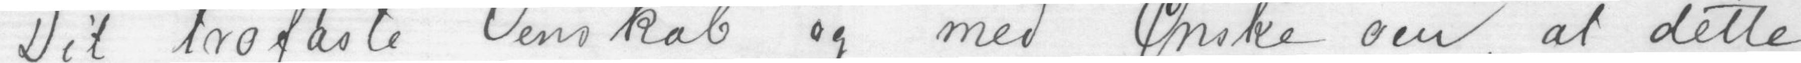Dit trofaste Venskab og med Ønske om, at dette
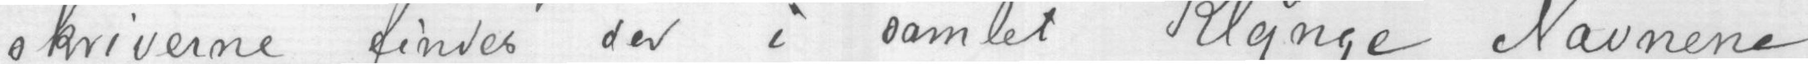skriverne findes der i samlet Klynge Navnene
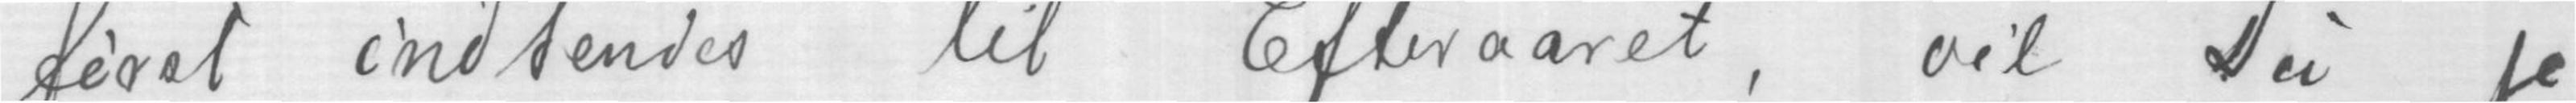først indtendes til Efteraaret, vil Du jo
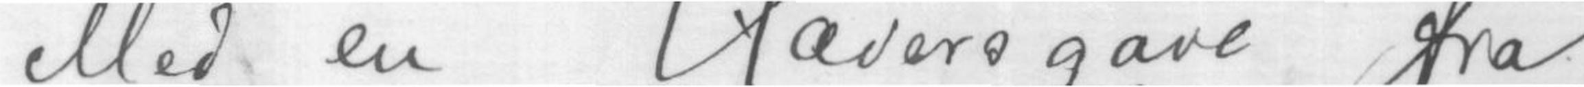Med en Hædersgave fra
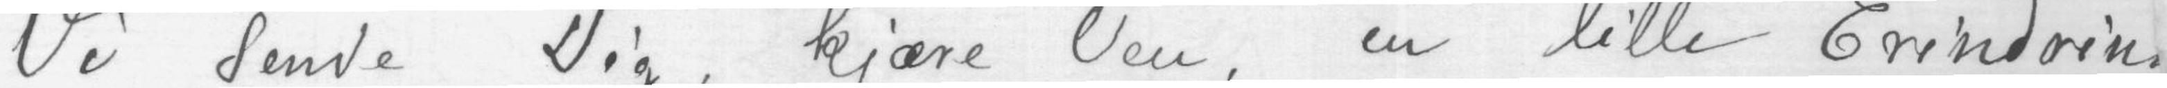Vi sende Dig, kjære Ven, en lille Erindring
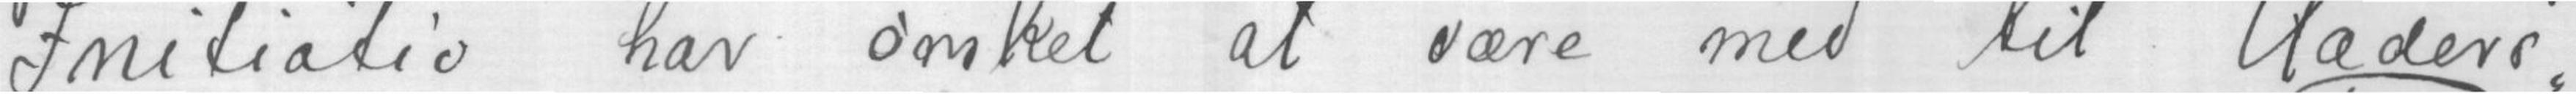Initiativ har ønsket at være med til Hæders-
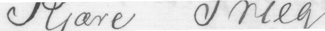Kjære Grieg!
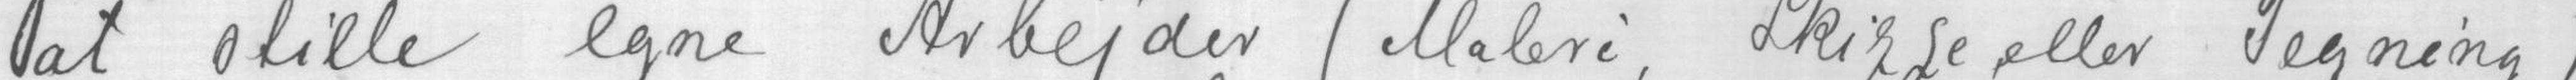at stille egne Arbejder (maleri, Skisse, eller Tegning)
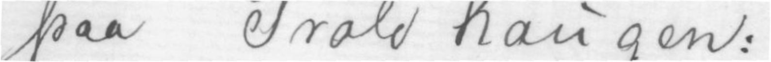paa Troldhaugen.-
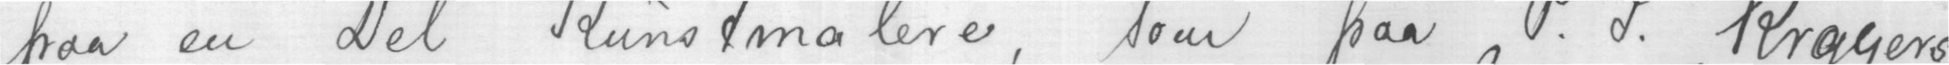paa en del Kunstmalere, som paa P.S.Krøyers
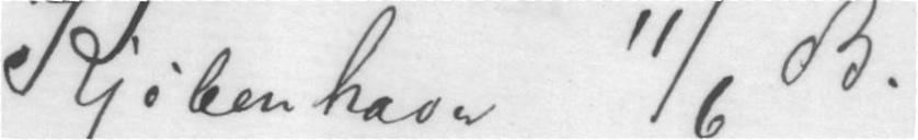Kjøbenhavn 11/6 03.
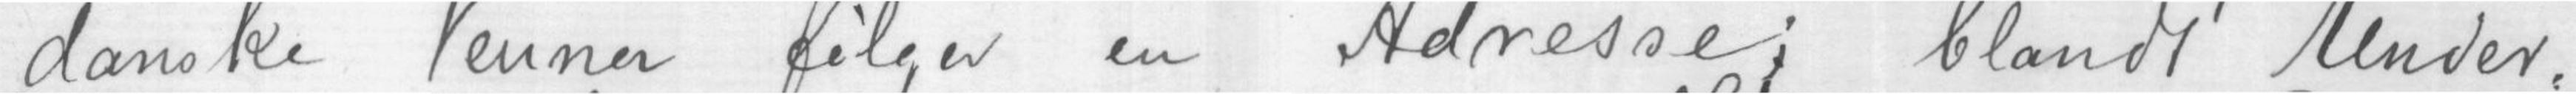danske Venner følger en Adresse blandt Under-
